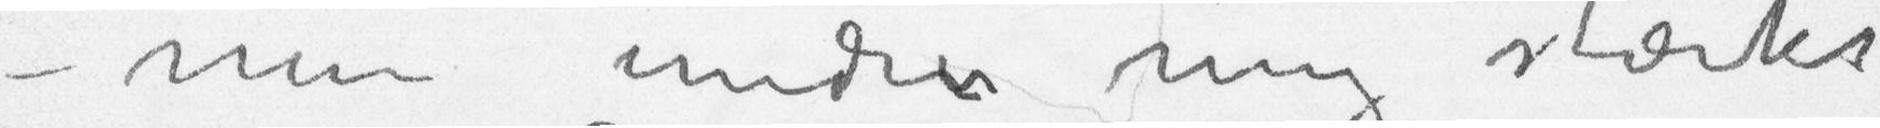– men undrer mig stærkt
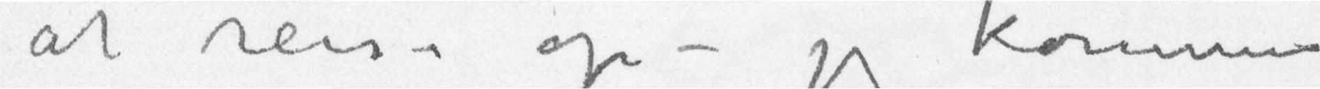at reise op – jeg kommer
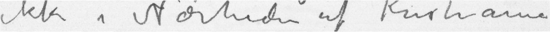ikke i Nærheden af Kristiania
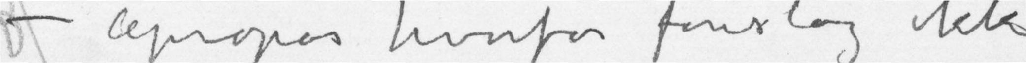– Apropos hvorfor foreslog ikke
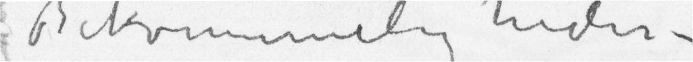Bekvemmeligheder –
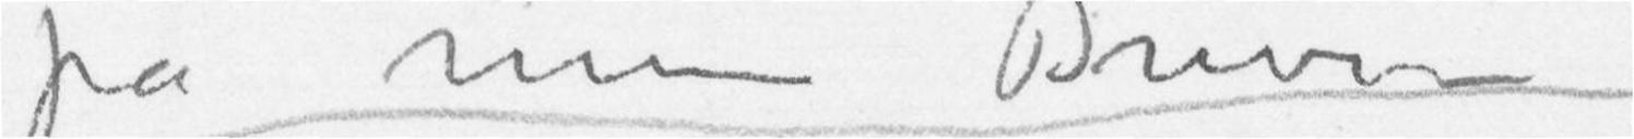på mine Breve
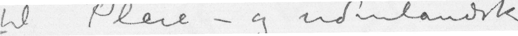til Pleie – og udenlandsk
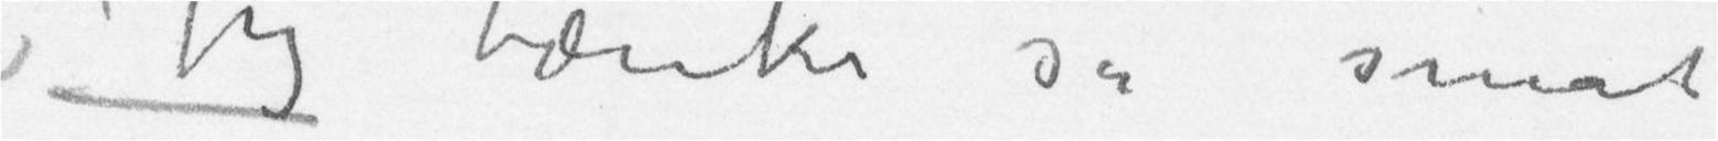Jeg tænker så småt
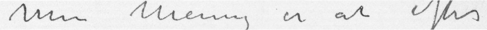Min Menig er at efter
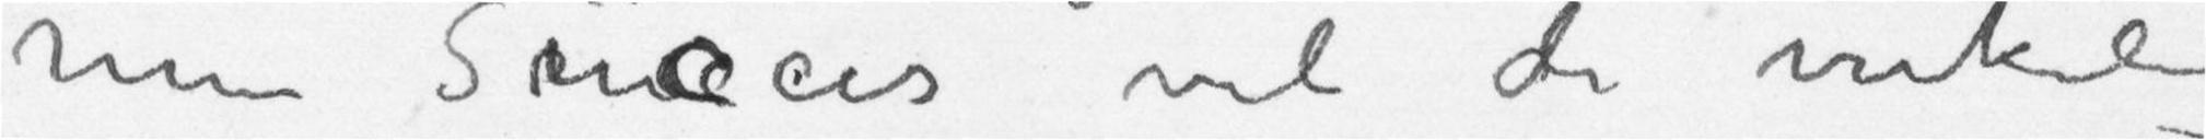min Sucess vil de virkelig
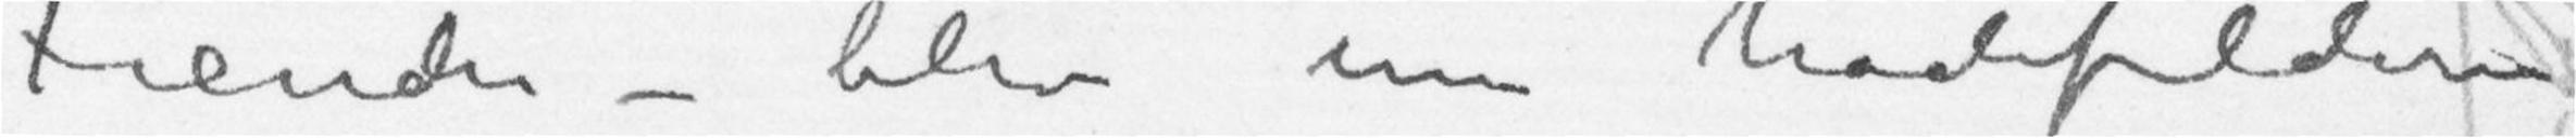Fiender – blive enn hadefuldere
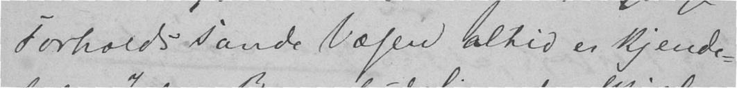Forholds sande Væsen altid er kjende-
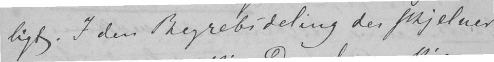ligt. I den Begrebsdeling der skjelner
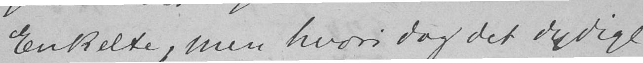Enkelte, men hvori dog det dydige
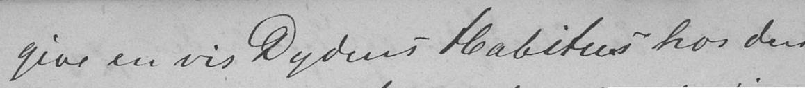giver en vis Dydens Habitus hos den
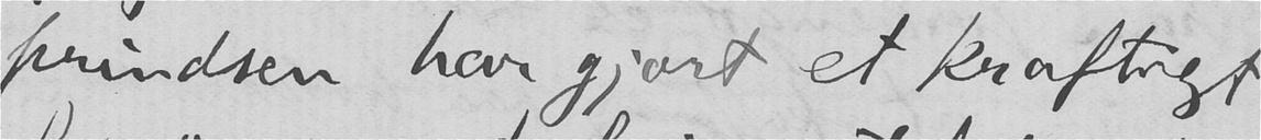prindsen har gjort et kraftigt
